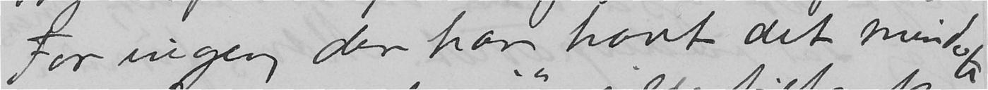For ingen der har havt det mindste
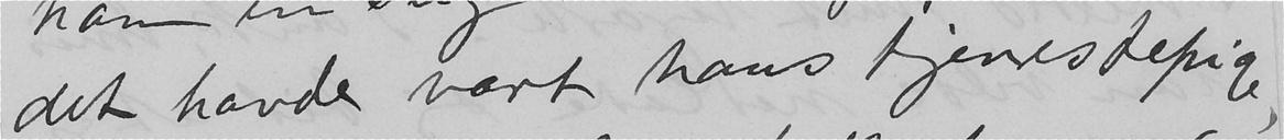det havde vært hans tjenestepige,
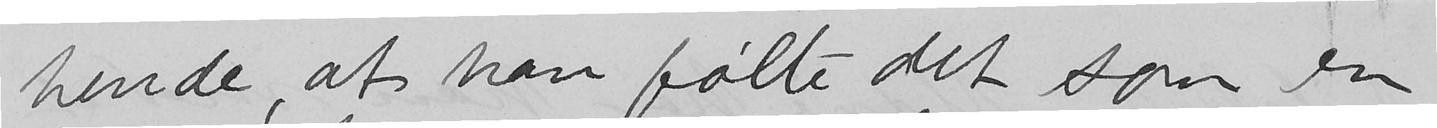hende, at han følte det som en
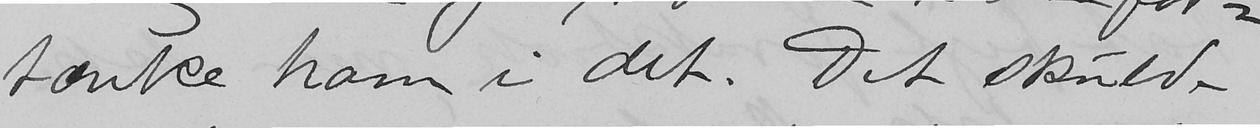tænke ham i det. Det skulde
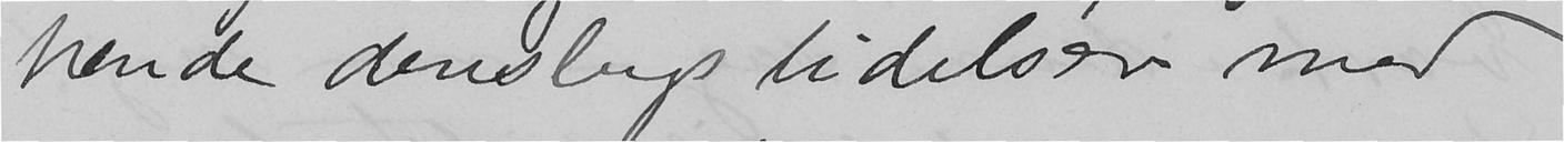hende denslags lidelser med
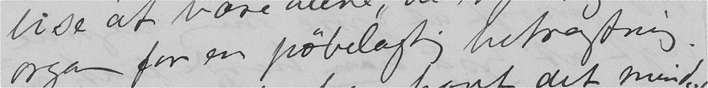organ for en pøbelagtig betragtning.
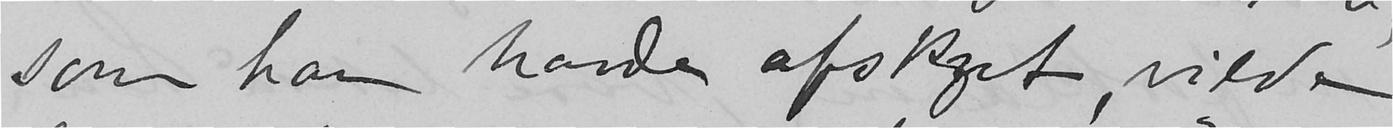som han havde afskyet, vilde
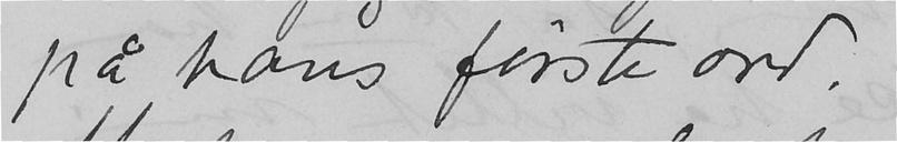på hans første ord.
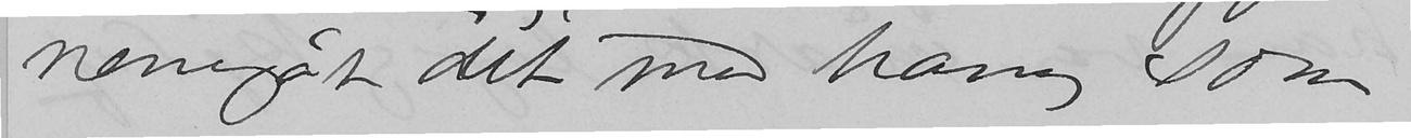nemgåt det med ham, som
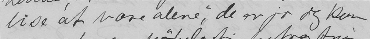lise at være alene", de er jo dog kun
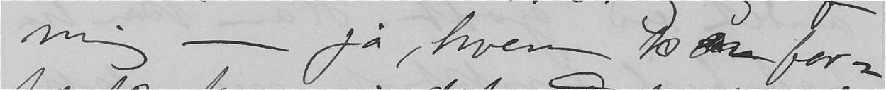mig - ja, hvem for-
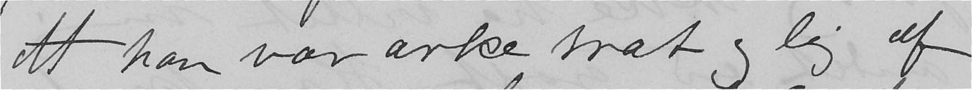At han var ærke træt og lej af
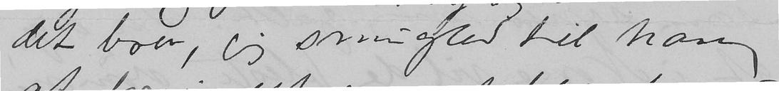det brev, jeg smugled til ham,
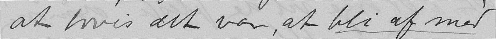at hvis det var, at bli af med
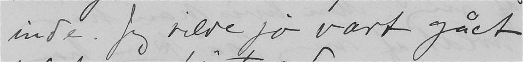inde. Jeg vilde jo vært gået
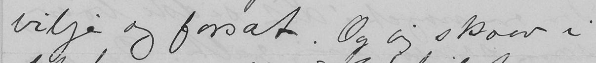vilje og forsæt. Og jeg skrev i
